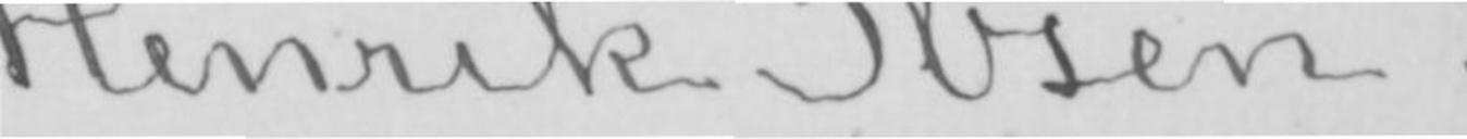Henrik Ibsen.
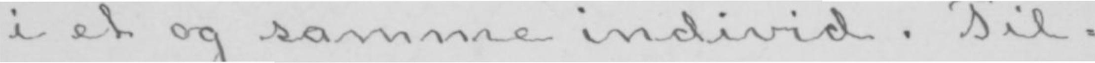i et og samme individ. Til-
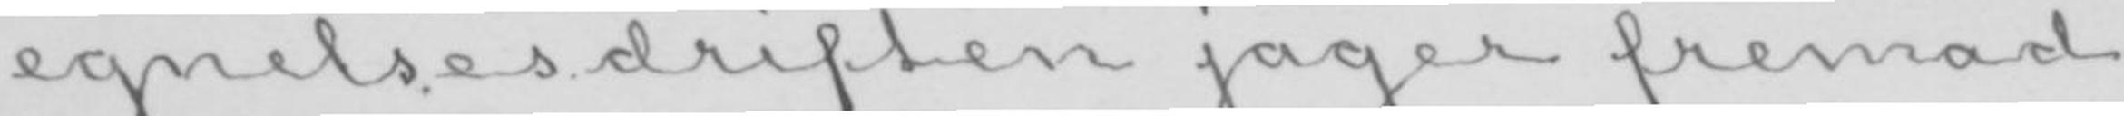egnelsesdriften jager fremad
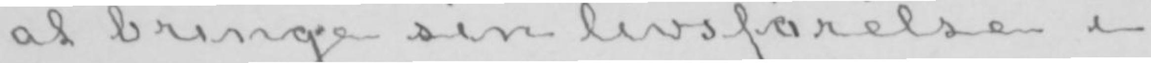at bringe sin livsførelse i
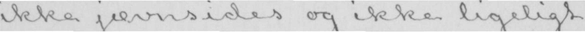ikke jævnsides og ikke ligeligt
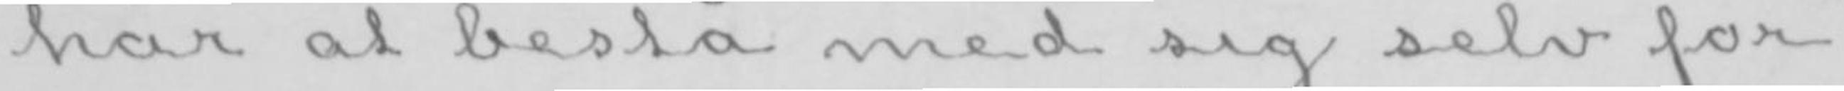har at bestå med sig selv for
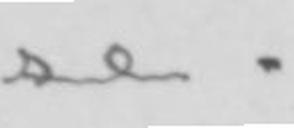se.
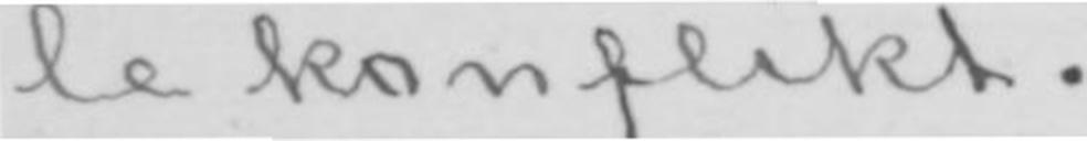le konflikt.
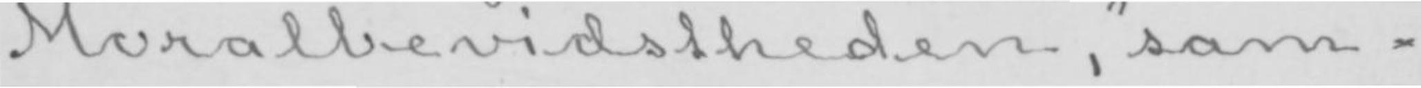Moralbevidstheden, «sam-
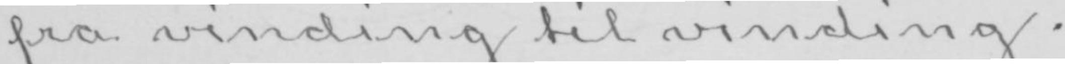fra vinding til vinding.
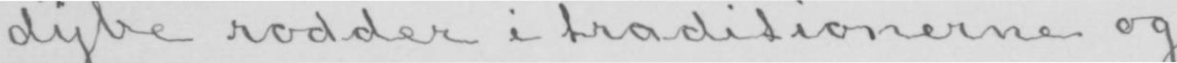dybe rødder i traditionerne og
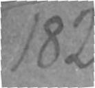182.
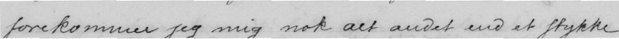forekommer jeg mig nok alt andet end et stykke
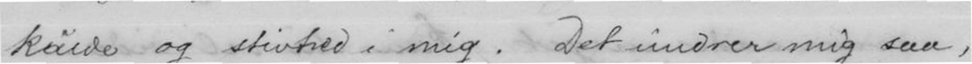kulde og stivhed i mig. Det undre mig saa,
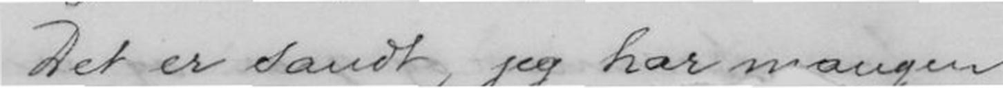Det er sandt, jeg har mangen
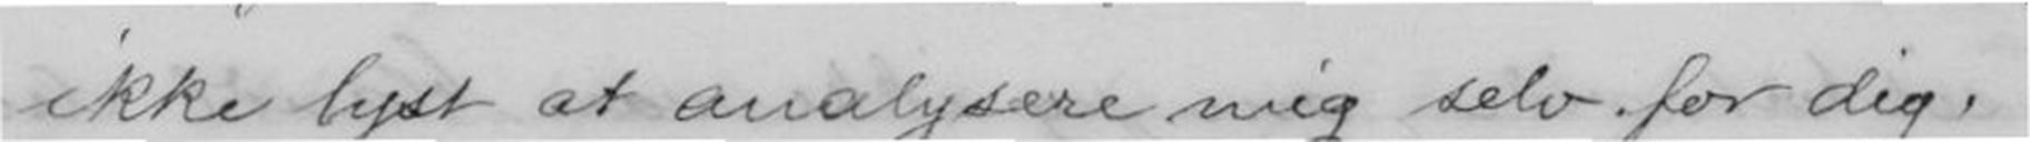ikke lyst at analysere mig selv for dig.
